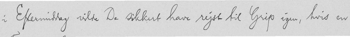i Eftermiddag vilde De sikkert have rejst til Grip igen, hvis en
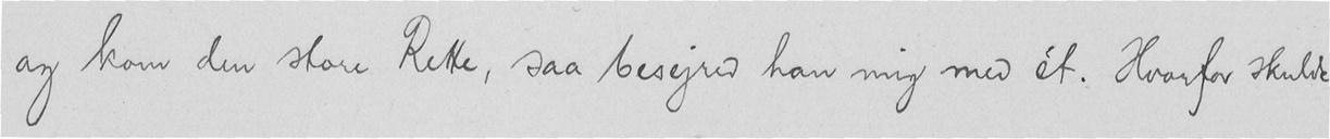og kom den store Rette, saa besejred han mig med ét. Hvorfor skulde
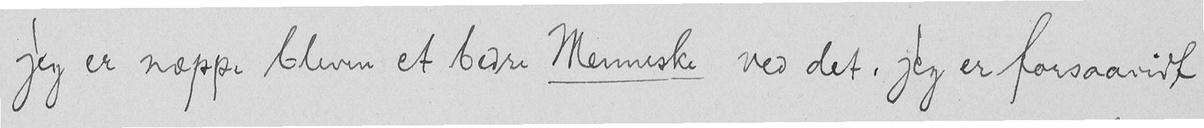jeg er næppe bleven et bedre Menneske ved det, jeg er forsaavidt
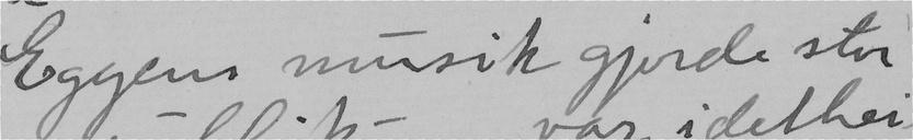Eggens musik gjorde stor
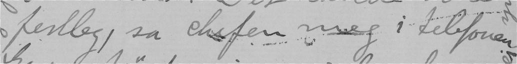festleg, sa chefen meg i telefonen.
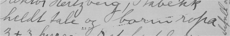heldt tale og born ropa
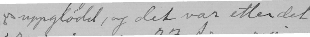uppglödd, og det var etter det
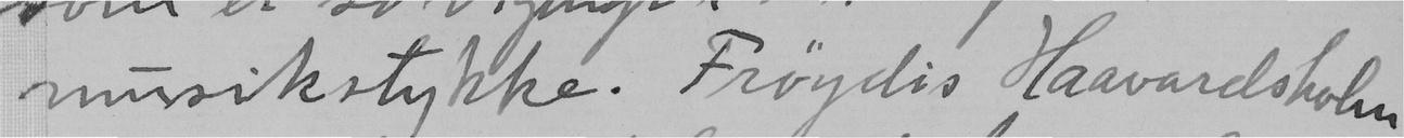musikstykke. Fröydis Haavardsholm
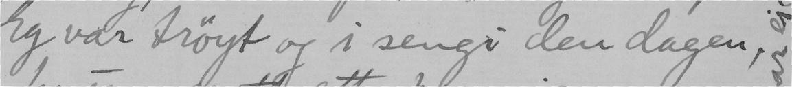Eg var tröyt og i sengi den dagen,
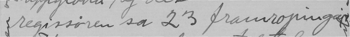regissören sa 23 framropingar.
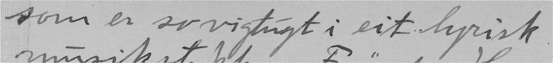som er so vigtugt i eit lyrisk
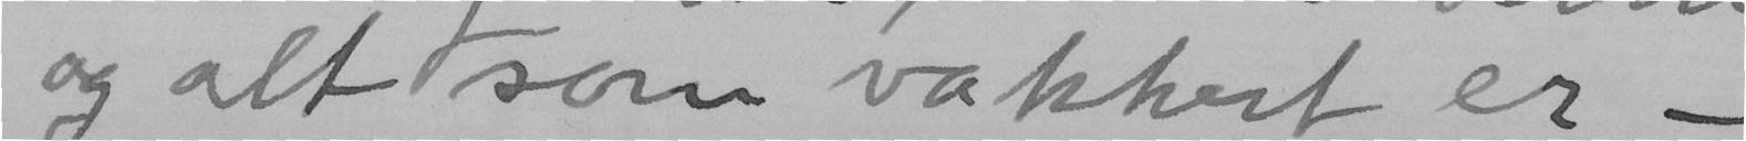og alt som vakkert er.
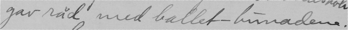gav råd med ballet-bunadene.
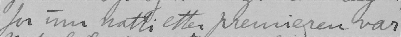for um natti etter premieren var
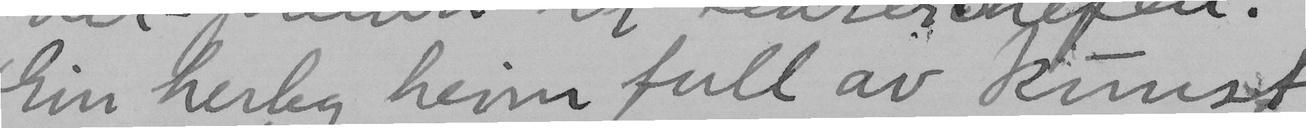Ein herleg heim full av kunst
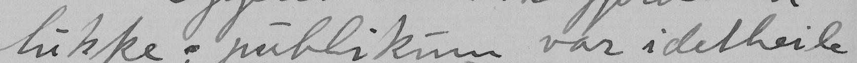lukke – publikum var i det heile
